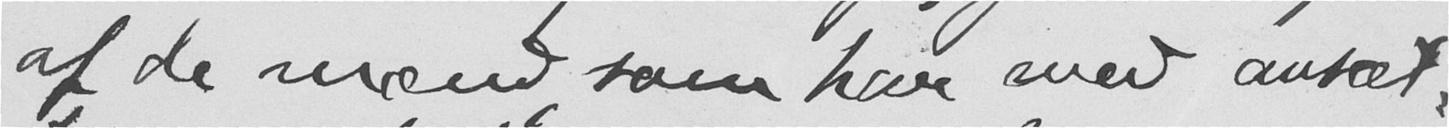af de mænd, som har med avsæt-
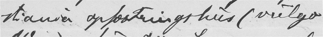stiania opfostringshus (vulgo
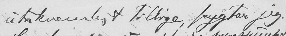utaknemligt tillige, frygter jeg.
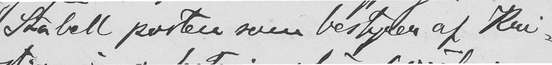Stabell posten som bestyrer af Kri-
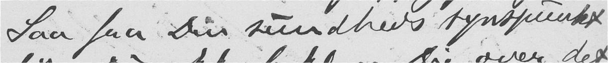Saa fra Din sundheds synspunkt
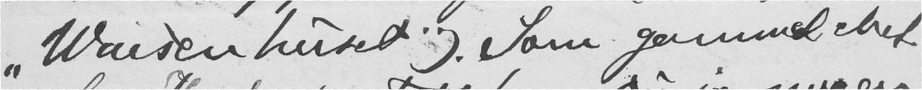"Waisenhuset"). Som gammel chef
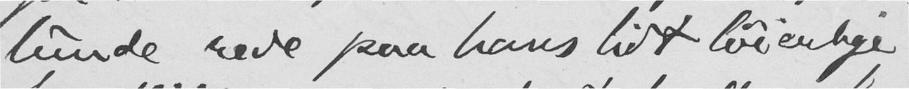lunde rede paa hans lidt løierlige
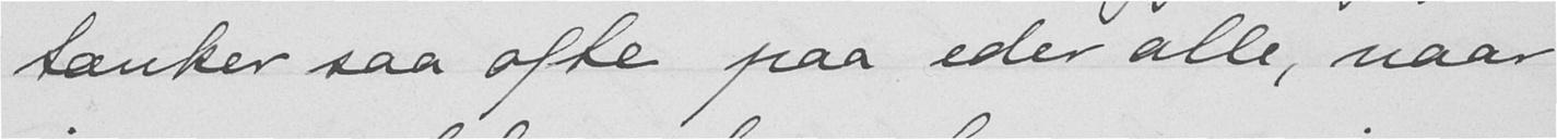tænker saa ofte paa eder alle, naar
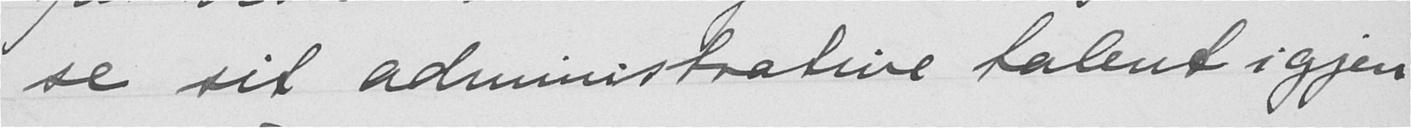se sit administrative talent igjen
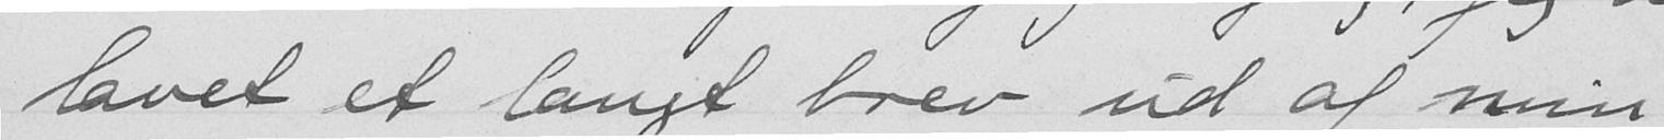lavet et langt brev ud af min
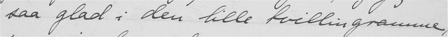saa glad i den lille tvillingramme,
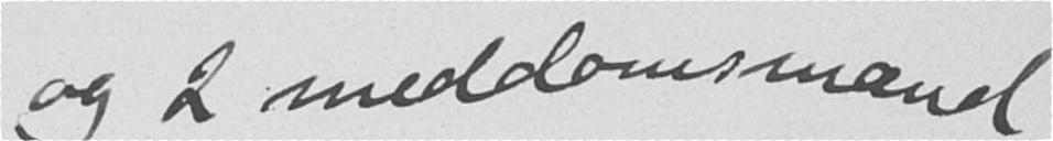og 2 meddomsmænd
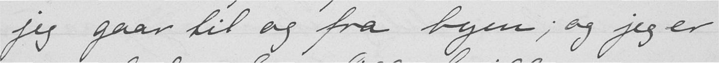jeg gaar til og fra byen; og jeg er
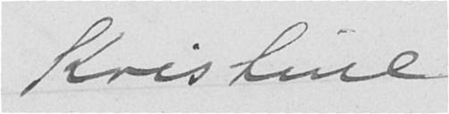Kristine.
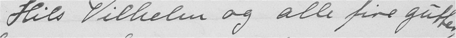Hils Vilhelm og alle fire gutter,
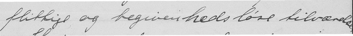flittige og begivenhedsløse tilværelse.
